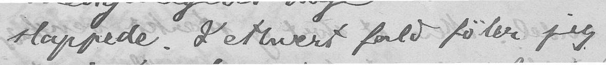stoppede. I ethvert fald føler jeg
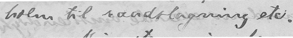holm til raadslagning etc.
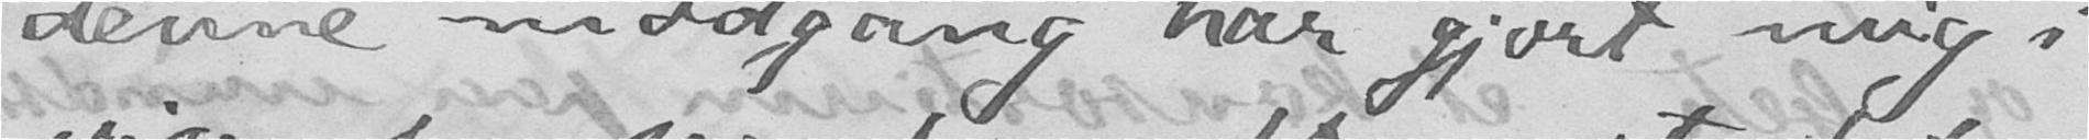denne modgang har gjort mig i
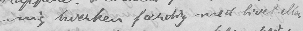mig hverken færdig med livet eller
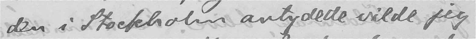den i Stockholm antydede vilde jeg
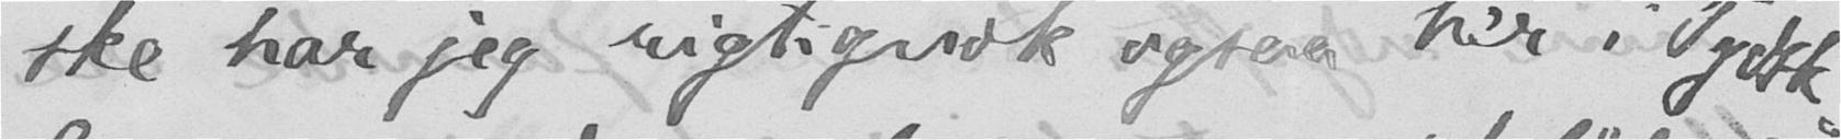ske har jeg rigtignok ogsaa her i Tydsk-
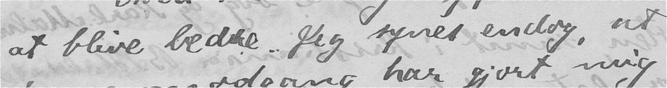at blive bedre. Jeg synes endog, at
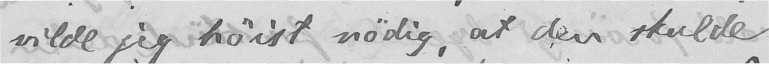vilde jeg høist nødig, at den skulde
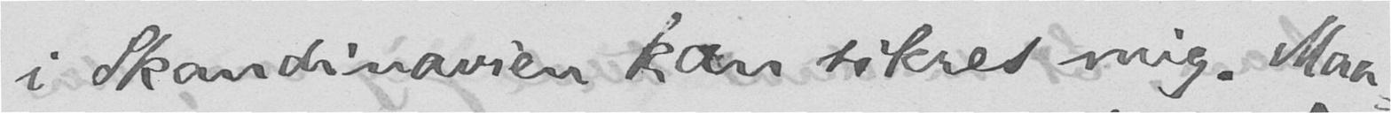i Skandinavien kan sikres mig. Maa-
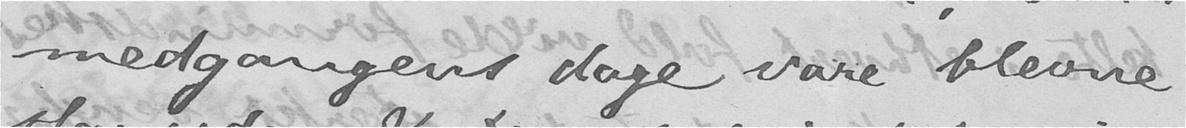medgangens dage vare blevne
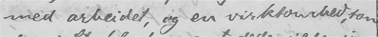med arbeidet, og en virksomhed, som
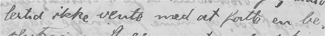lertid ikke vente med at fatte en be-
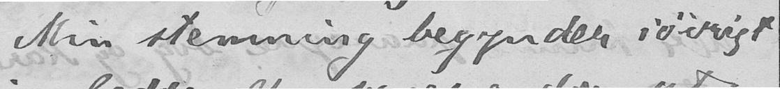Min stemning begynder iøvrigt
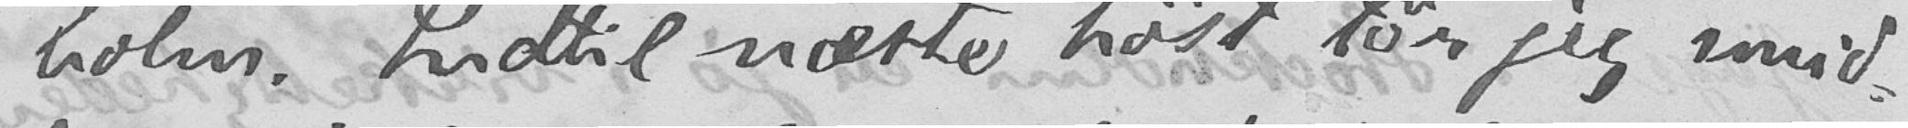holm. Indtil næste høst tør jeg imid-
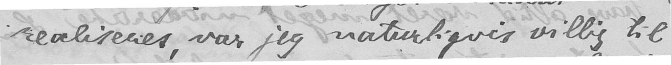realiseres, var jeg naturligvis villig til
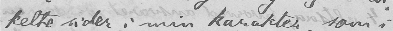kelte sider i min karakter, som i
